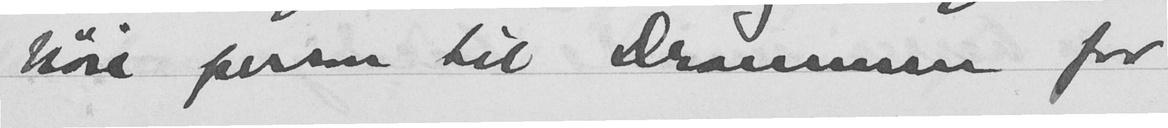høie person til Drammen for
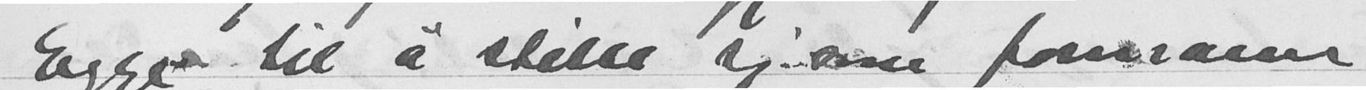Egge til å stille sig som formann
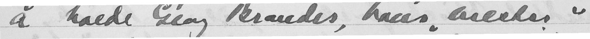å holde Georg Brandes, hans "mester"
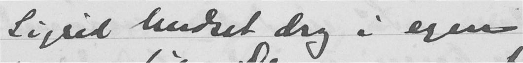Sigrid Undset drog i egen
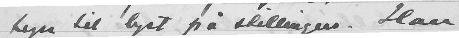tegn til lyst på stillingen. Han
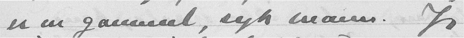er en gammel, syk man. Jeg
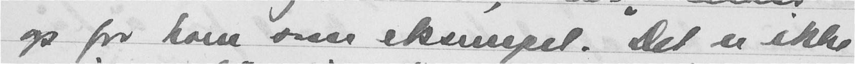op for ham som eksempel. Det er ikke
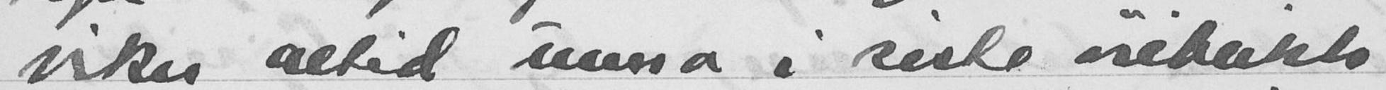viker alltid unna i siste øieblikk
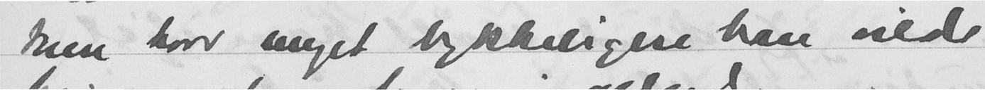Men hvor meget lykkeligere han vilde
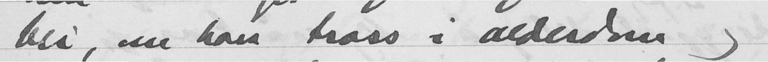bli, om han tross i alderdom og
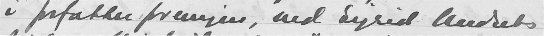i forfatterforeningen, ved Sigrid Undsets
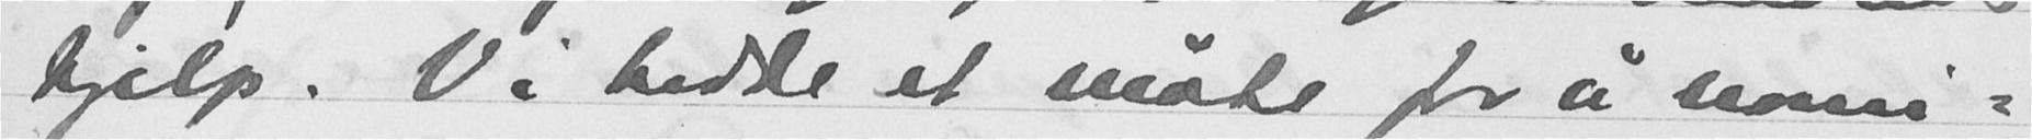hjelp. Vi hadde et møte for a nomi-
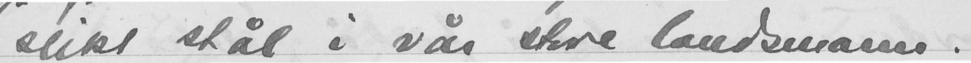slikt stål i vår store landsmann.
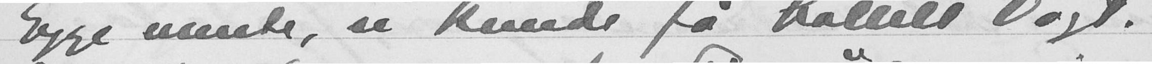Egge mente, vi kunde få Collett Vogt.
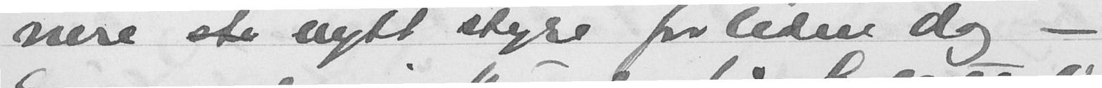nere  nytt styre forleden dag -
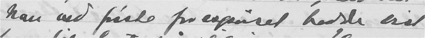han ved første forespørsel hadde vist
